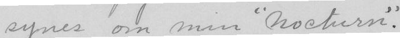synes om min "Nocturn".
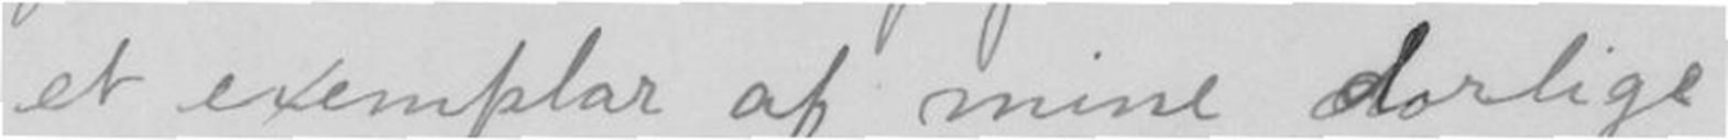et exemplar af mine Aarlige
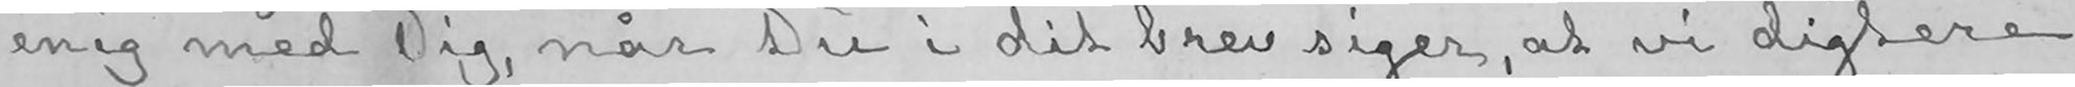enig med Dig, når Du i dit brev siger, at vi digtere
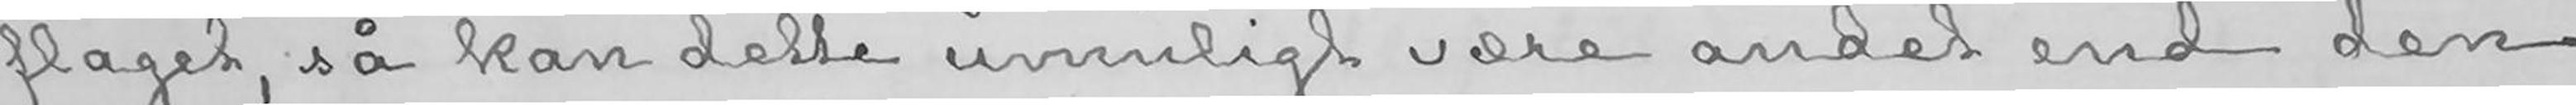flaget, så kan dette umuligt være andet end den
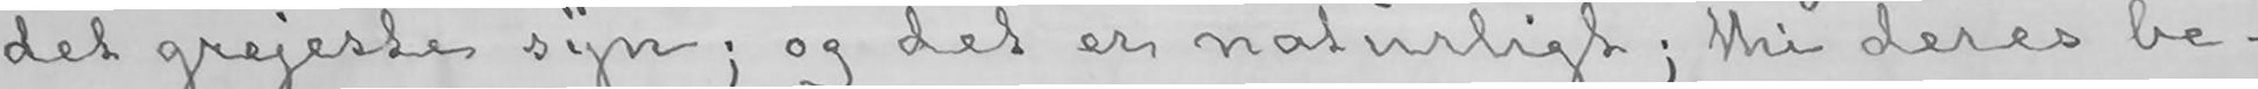det grejeste syn; og det er naturligt; thi deres be-
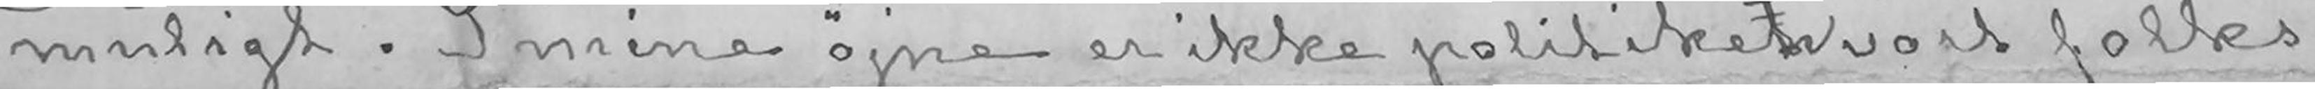muligt. I mine øjne er ikke politiken vort folks
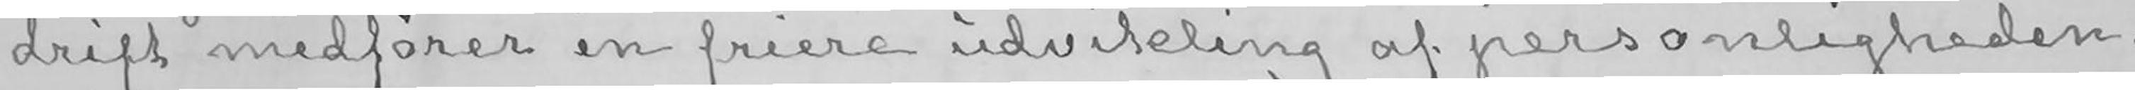drift medfører en friere udvikling af personligheden.
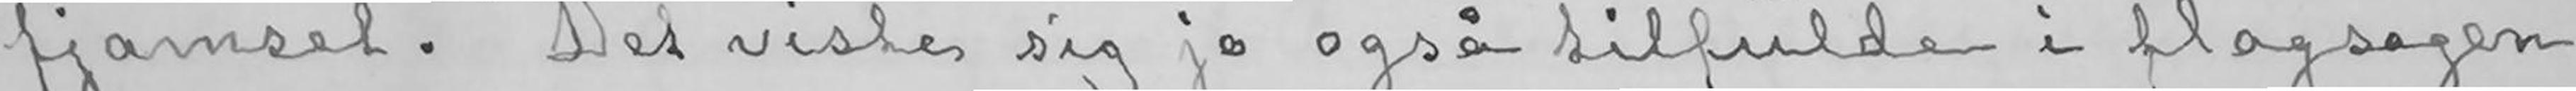fjamset. Det viste sig jo også tilfulde i flagsagen,
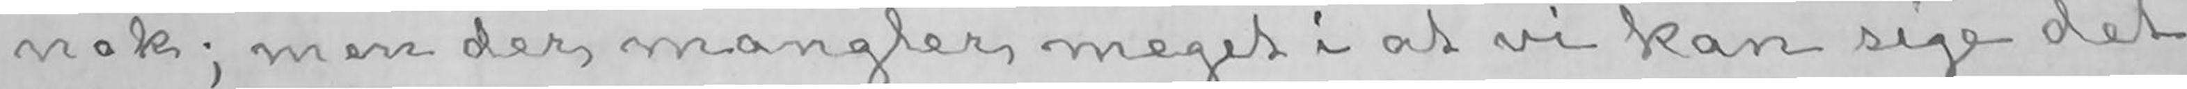nok; men der mangler meget i at vi kan sige det
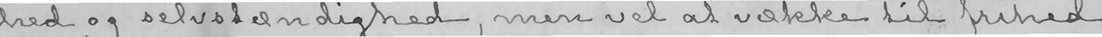hed og selvstændighed, men vel at vække til frihed
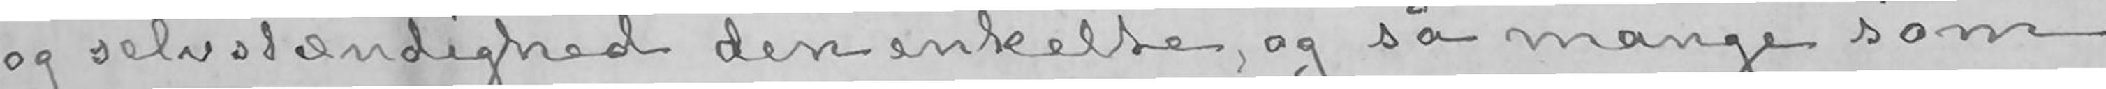og selvstændighed den enkelte, og så mange som
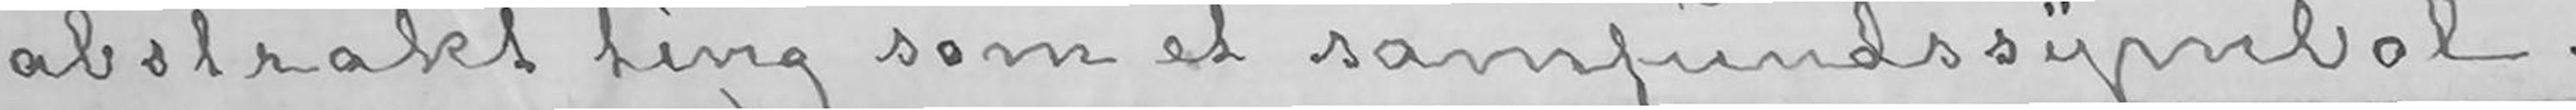abstrakt ting som et samfundssymbol.
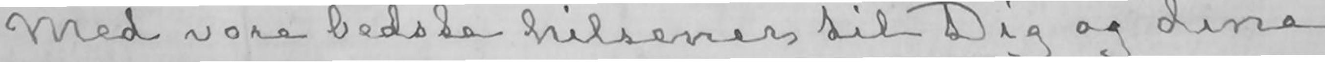Med vore bedste hilsener til Dig og dine
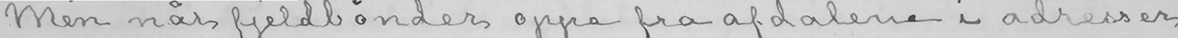Men når fjeldbønder oppe fra afdalene i adresser
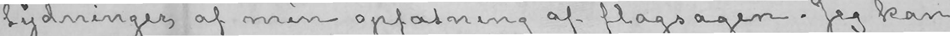tydninger af min opfatning af flagsagen. Jeg kan
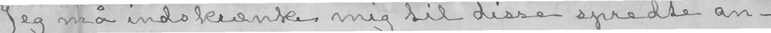Jeg må indskrænke mig til disse spredte an-
